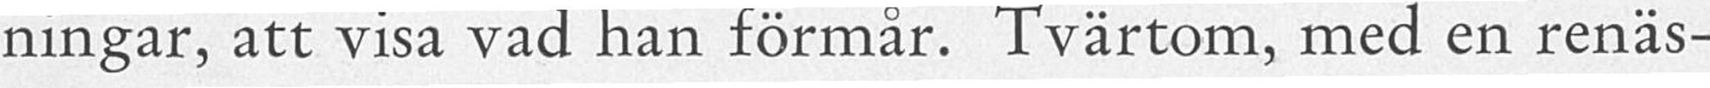ningar, att visa vad han förmår. Tvärtom, med en renäs-
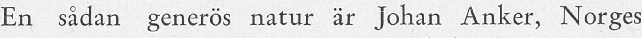En sådan generös natur är Johan Anker, Norges
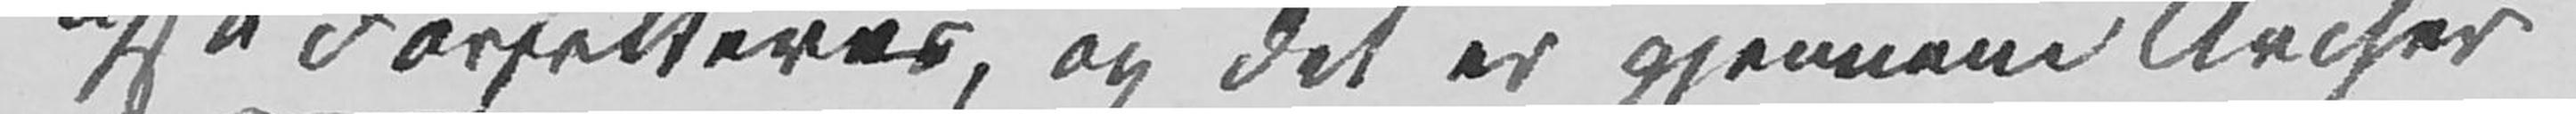også Forfatteres, og det er gjennem Aviser
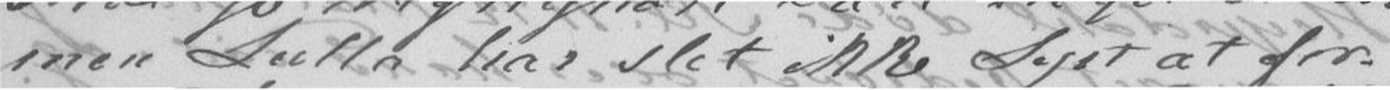men Lulla har slet ikke Lyst at for-
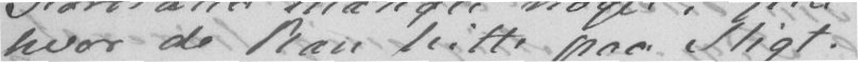hvor de kan hitte paa Sligt.
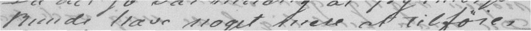kunde have noget mere at tilføie,
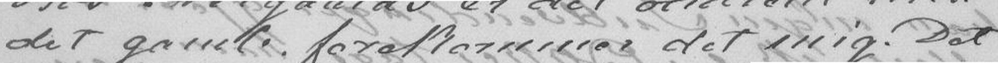det gamle forekommer det mig. Det
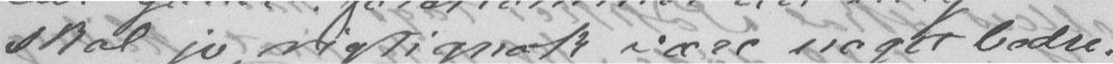skal jo rigtignok være noget bedre.
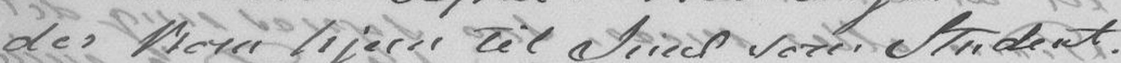der kom hjem til Juul som Student,
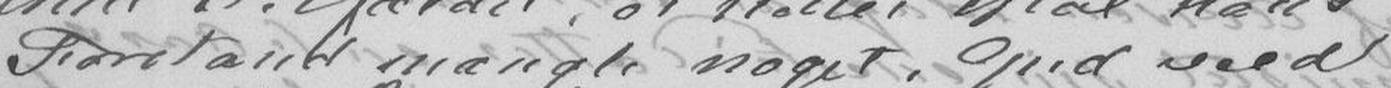Forstand mangle noget, Gud veed
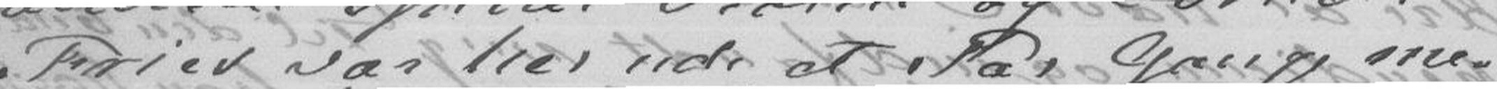Fries var her ude et Par Gange me-
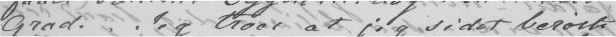Grad. Jeg troer at jeg sidst berørte
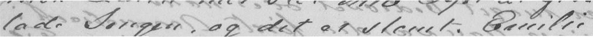lade Sengen, og det er slemt. Emilie
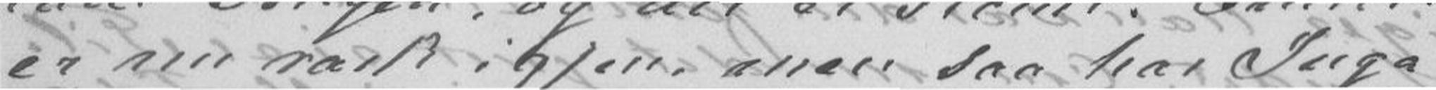er nu rask igjen, men saa har Inga
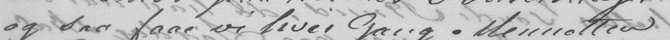og saa faae vi hver Gang Menuetten
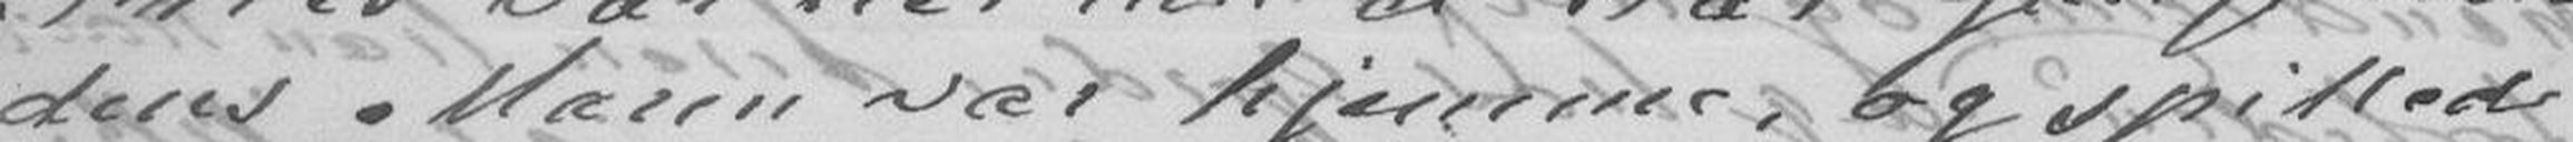dens Maren var hjemme, og spillede
